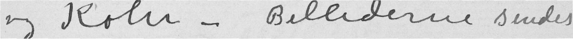og Köln – Billederne sendes
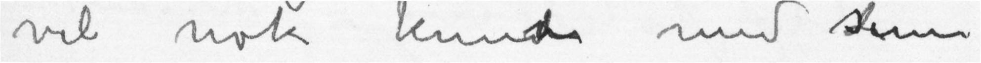vil nok kunde med sine
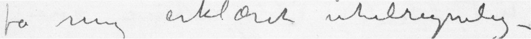få mig erklært utilregnelig –
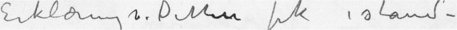Erklæring v. Ditten fik i stand –
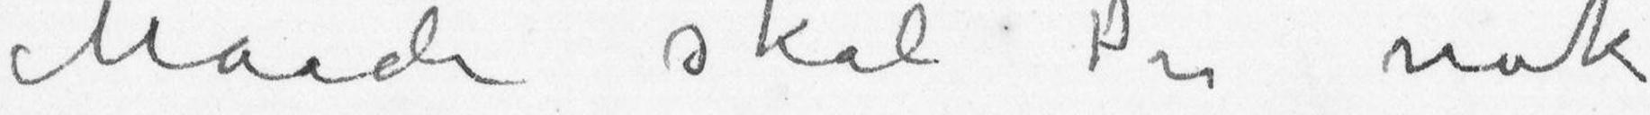Maade skal Du nok
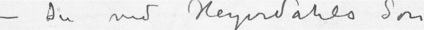– Du ved Heyerdahls Søn
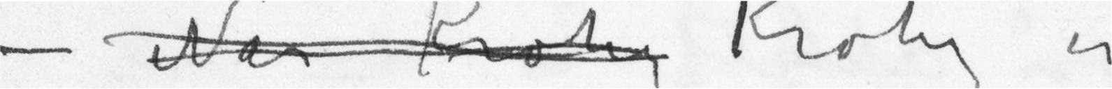– Når Krohg Krogh er
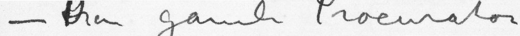– Den gamle Provocatør
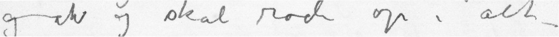og ik og skal rode op i alt –
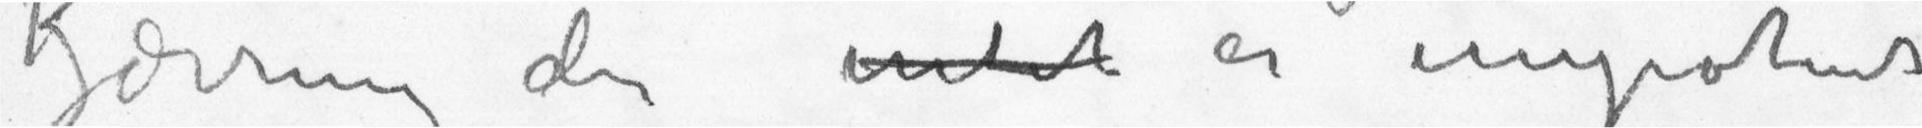Kjærring der intet er impotent
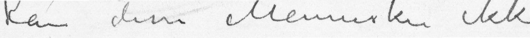Kan disse Mennesker ikke
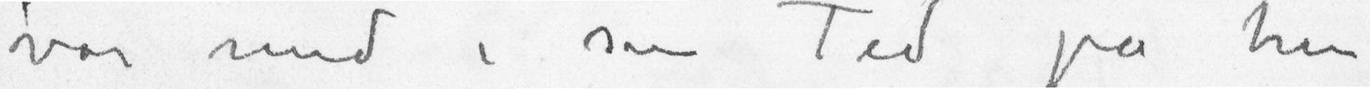var med i sin Tid på hin
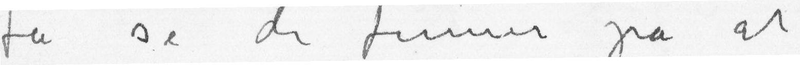få se de finner på at
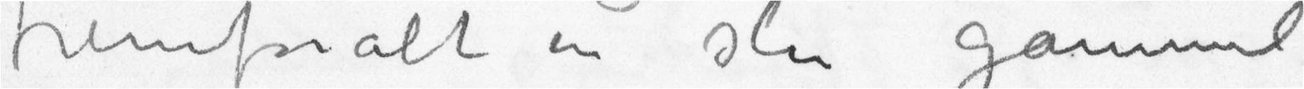fremforalt en slu gammel
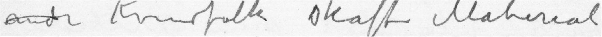andre Kvindfolk skaffe Material
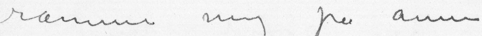ramme mig på annen
